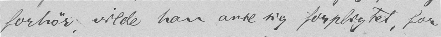forhør, vilde han anse sig forpligtet, for
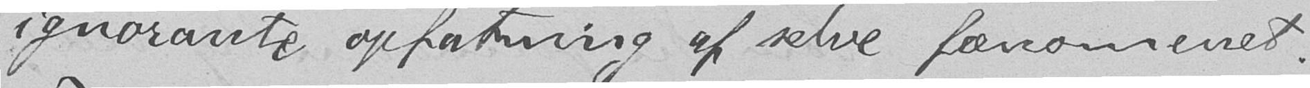ignorante opfatning af selve fænomenet.
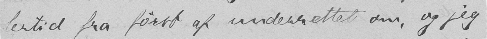lertid fra først af underrettet om, og jeg
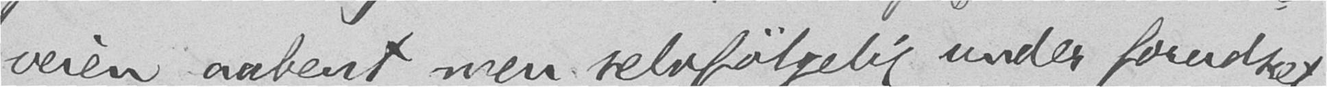veien aabent, men selvfølgelig under forudset-
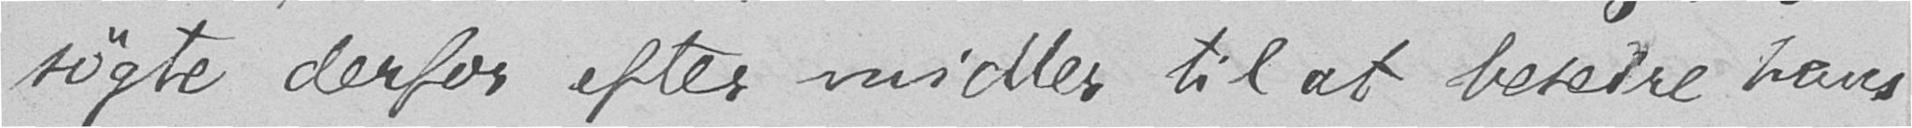søgte derfor efter midler til at beseire hans
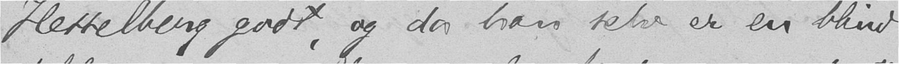Hesselberg godt, og da han selv er en blind
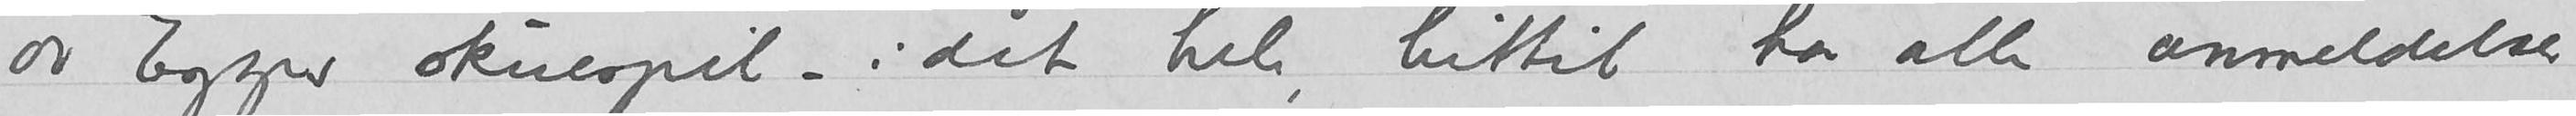av Egges skuespil – i det hele, hittil har alle anmeldelser
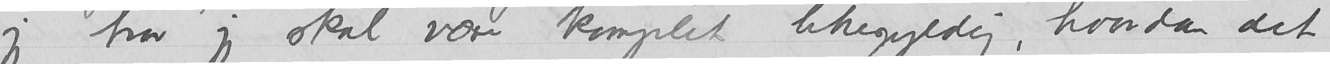– jeg tror jeg skal være komplet likegyldig, hvordan det
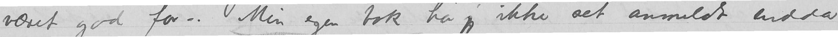været god for -. Min egen bok har jeg ikke set anmeldt endda
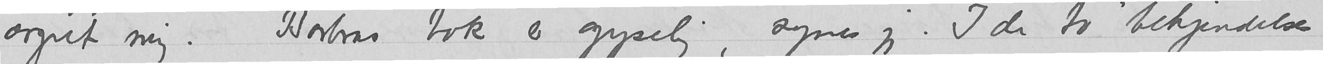ærgret mig. Barbras bok er gyselig, synes jeg. I de to «bekjendelses-
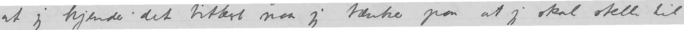at jeg kjender det bittert naar jeg tænker paa at jeg skal stelle til
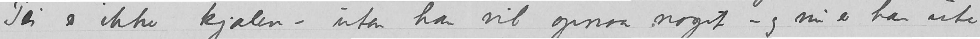Pei er ikke kjælen – uten han vil opnaa noget – og nu er han ute
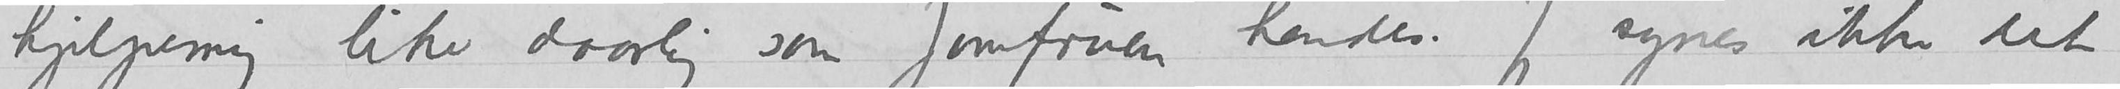hjelpemig like daarlig som Jomfruen hendes. Jeg synes ikke det
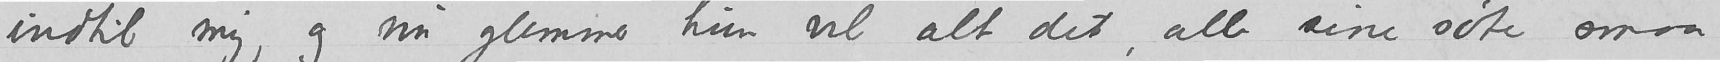indtil mig, og nu glemmer hun vel alt det, alle sine søte smaa
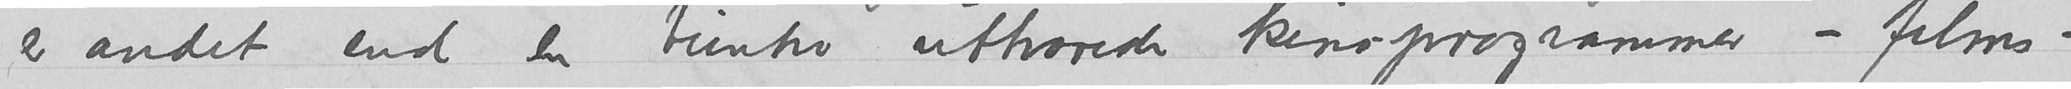er andet end en bunke uttværede kinoprogrammer – films-
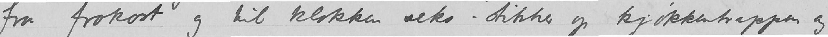fra frokost og til klokken seks – stikker op kjøkkentrappen og
